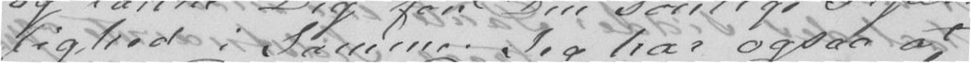lighed i Sommer. Jeg har ogsaa at
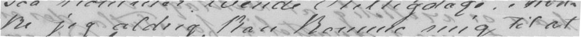ke jeg aldrig kan komme mig til at
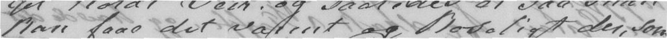kan faae det varmt og koseligt der, som
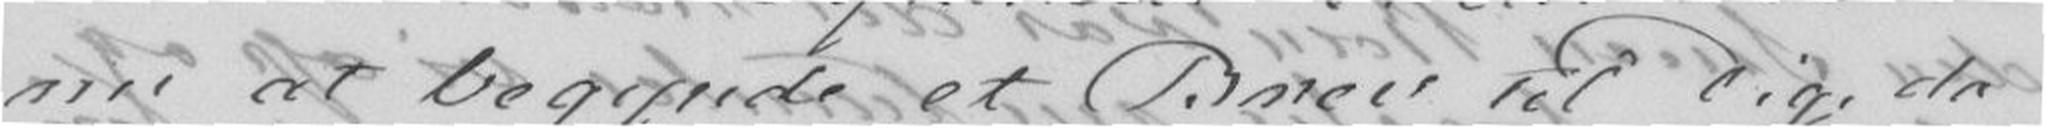nu at begynde et Brev til dig da
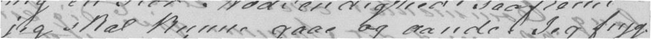jeg skal kunne gaae og aande. Jeg fryg-
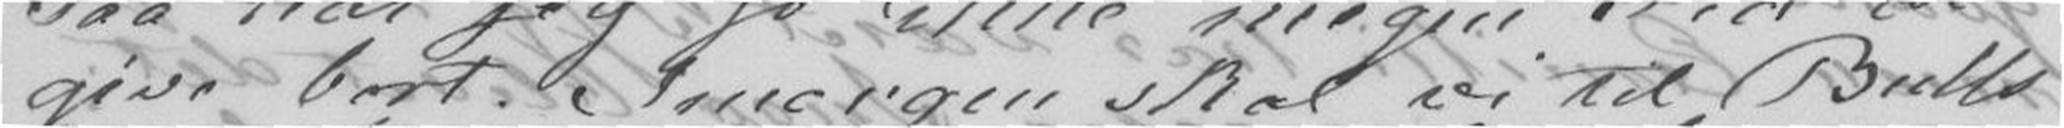give bort. Imorgen skal vi til Bulls
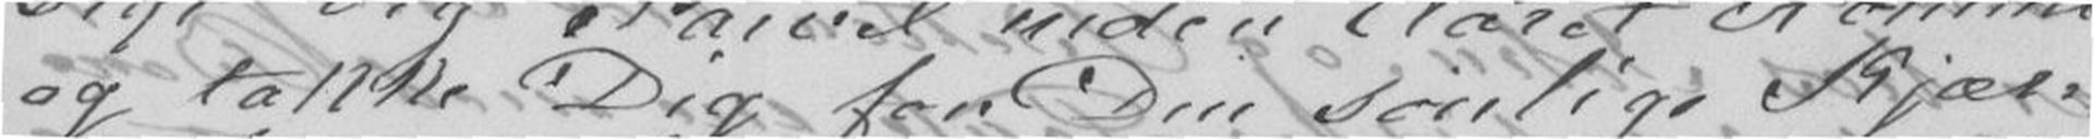og takke Dig for Din sønlige Kjær-
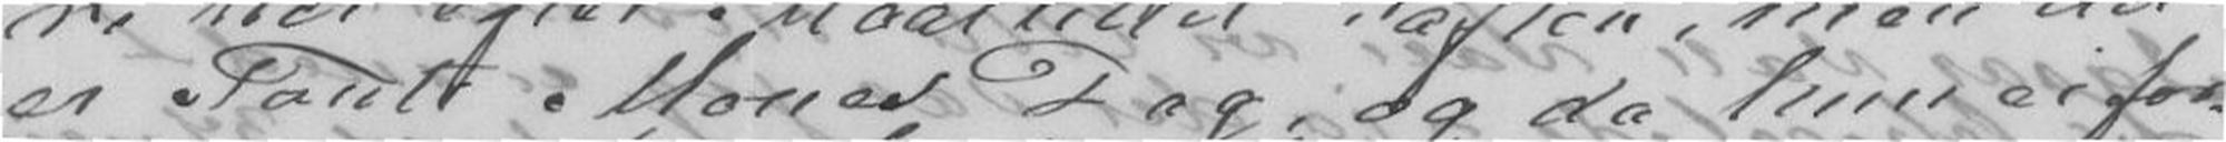er Tante Mones Dag, og da hun ei for-
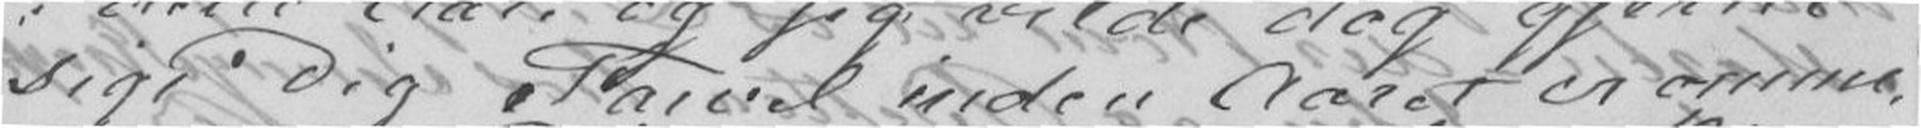sige Dig Farvel inden Aaret er omme
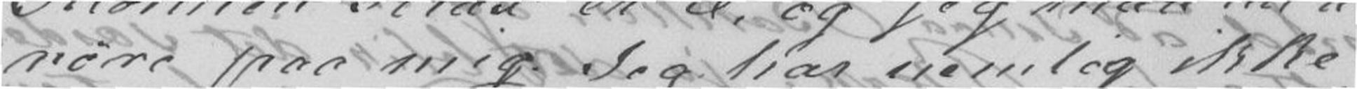røre paa mig. Jeg har nemlig ikke
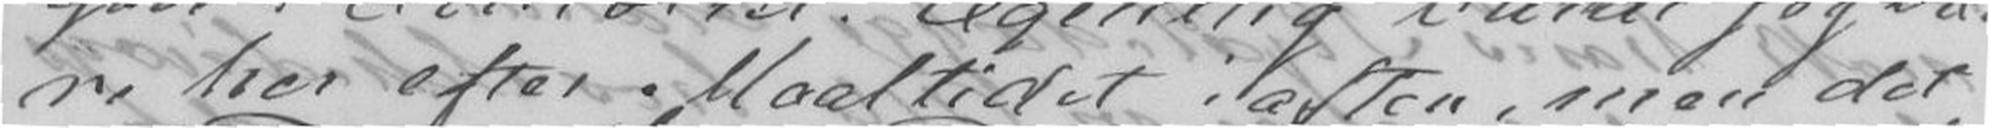re her efter Maaltidet iaften, men det
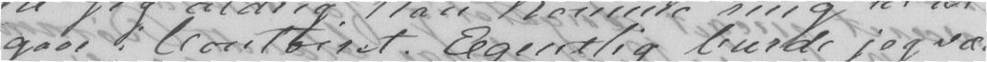gaae i Contoiret. Egentlig burde jeg væ-
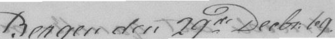Bergen den 29de Decbr 69.
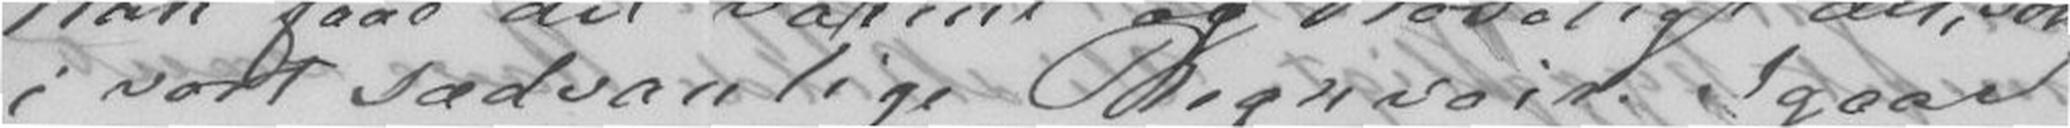i vort sædvanlige Regnveir Igaar
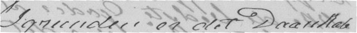Igrunden er det Daarskab
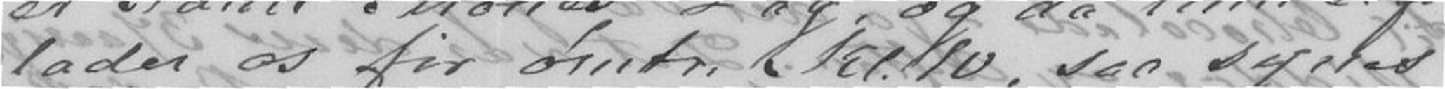lader os før omtr. Kl.10, saa synes
